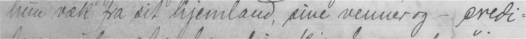hun væk fra sit hjemland, sine venner og - credi-
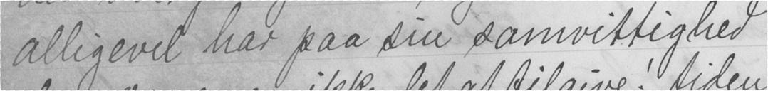alligevel har paa sin samvittighed
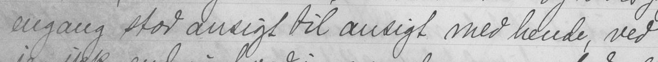engang stod ansigt til ansigt med hende, ved
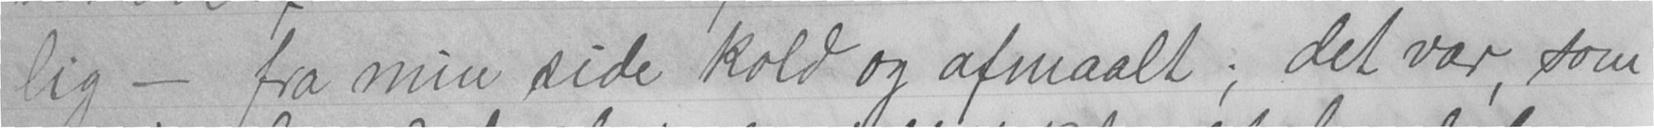lig - fra min side kold og afmaalt; det var, som
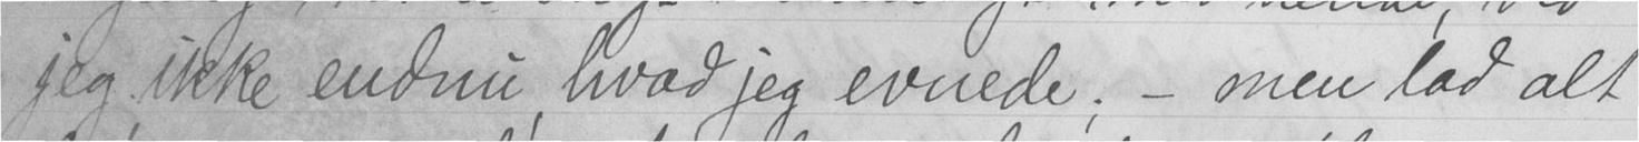jeg ikke endnu, hvad jeg evnede; - men lad alt
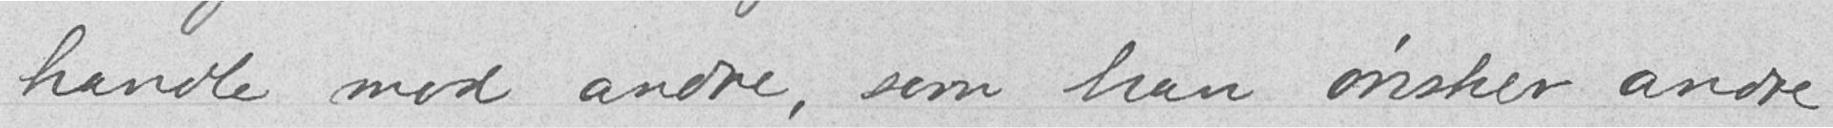handle mod andre, som han ønsker andre
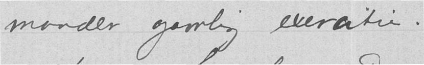maader gavnlig exercitie.
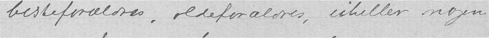besteforældres, oldeforældres, eiheller nogen
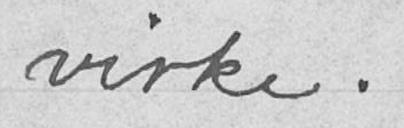virke.
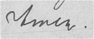Amen.
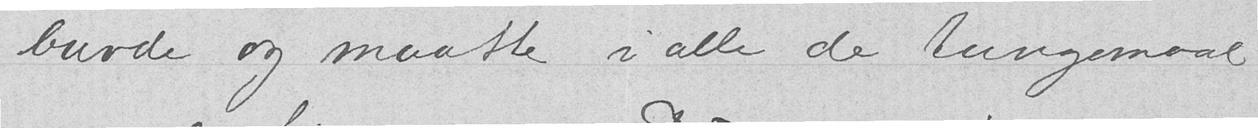burde og maatte i alle de tungemaal
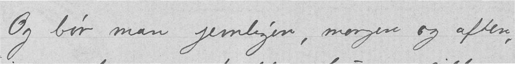Og bør man jevnligen, morgen og aften,
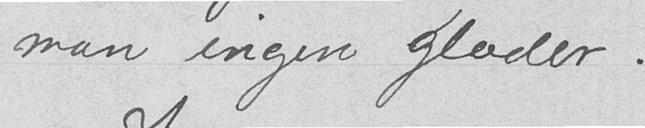man ingen glæder.
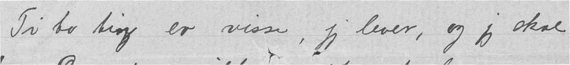Ti to ting er visse, jeg lever, og jeg skal
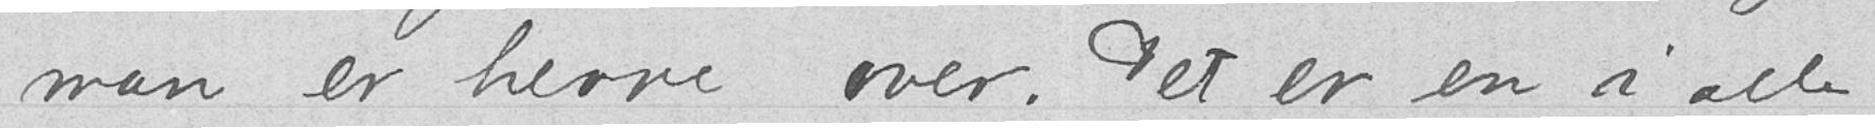man er herre over. Det er en i alle
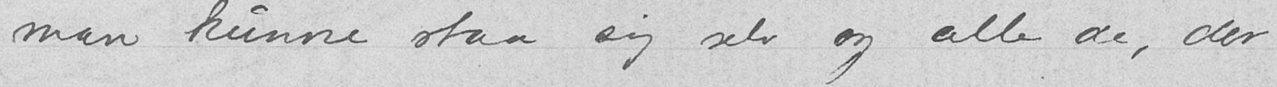man kunne staa sig selv og alle de, der
